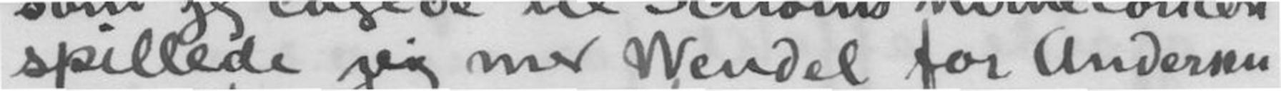spillede jeg med Wendel for Andersen
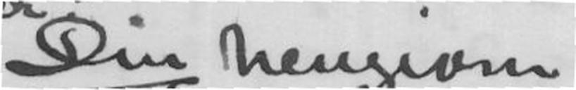Din hengivne
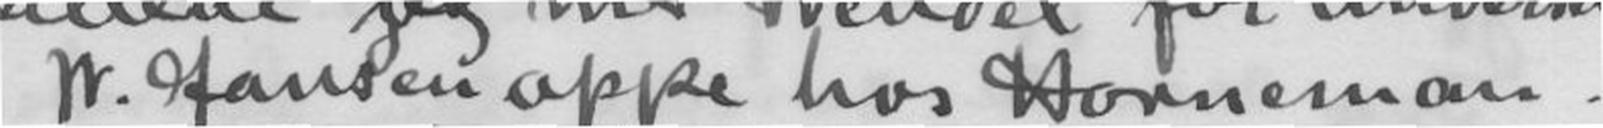og W. Hansen oppe hos Horneman.
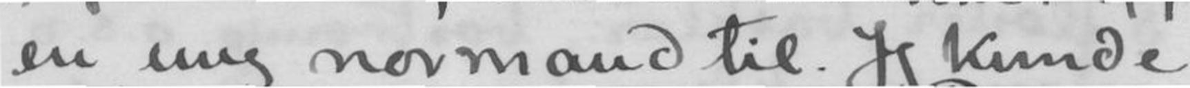en ung normand til. Jeg kunde
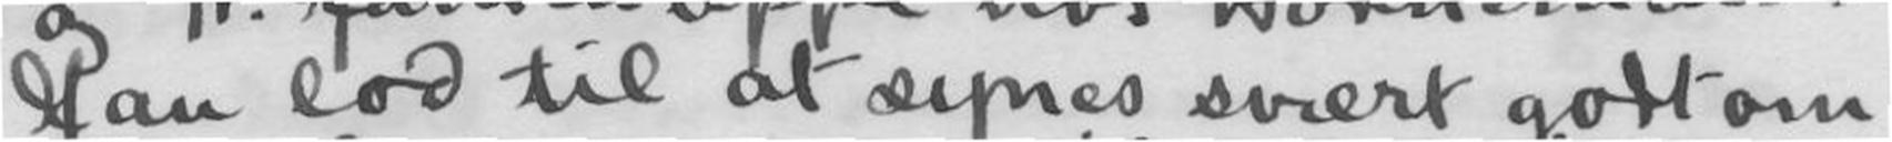Han lod til at synes svært godt om
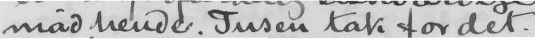mod hende. Tusen tak for det.
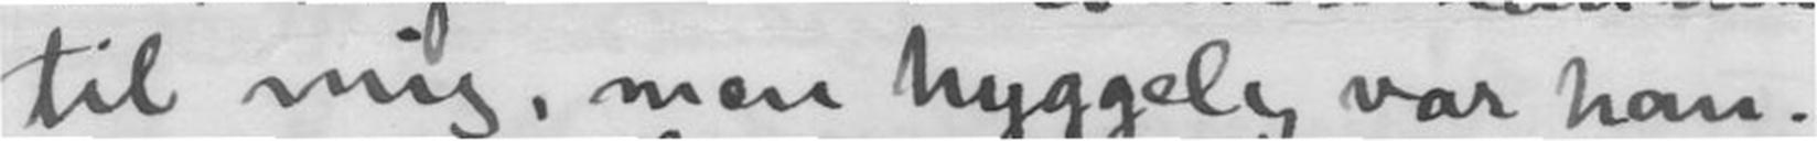til mig, men hyggelig var han.
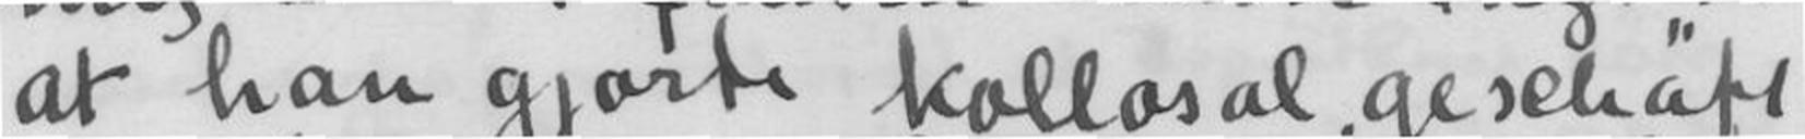at han gjorde kollosal, geschäft
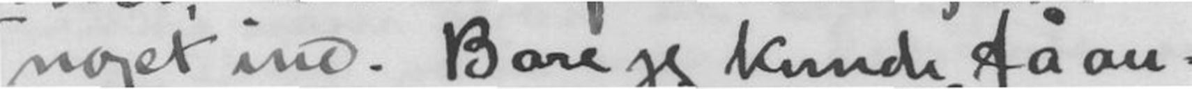noget ind. Bare jeg kunde få an-
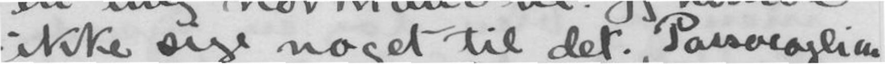ikke sige noget til det. Passacaglian
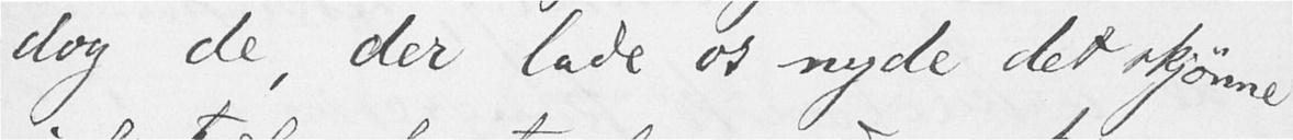dog de, der lade os nyde det skjønne
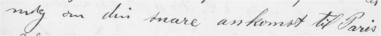mig om din snare ankomst til Paris.
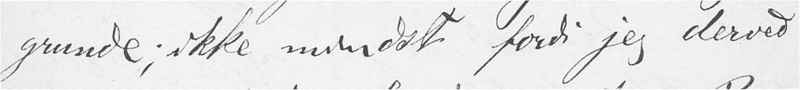grunde; ikke mindst fordi jeg derved
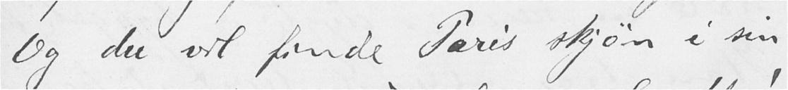Og du vil finde Paris skjøn i sin
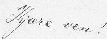Kjære ven!
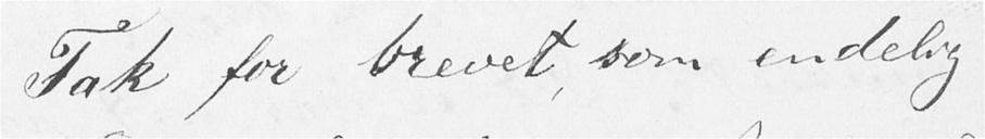Tak for brevet, som endelig
